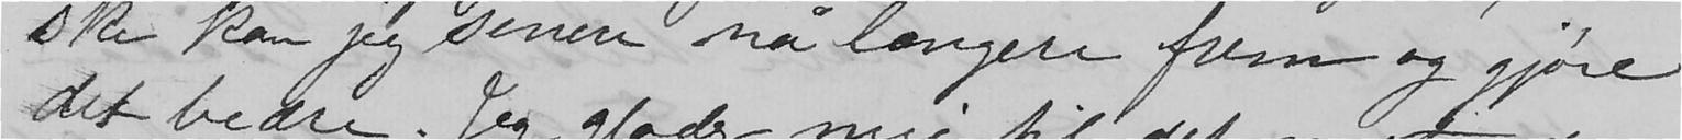ske kan jeg senere nå længere frem og gjøre
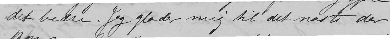det bedre. Jeg glæder mig til det næste der
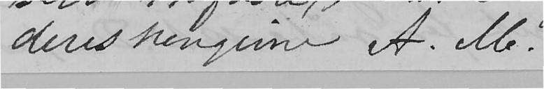deres hengivne A.M.
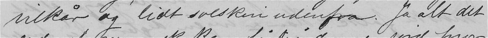vilkår og lidt solskin udenfra. Ja alt det
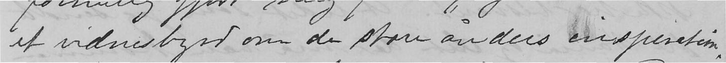et vidnesbyrd om de store ånders inspiration.
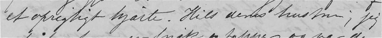et oprigtigt hjærte. Hils deres hustru; jeg
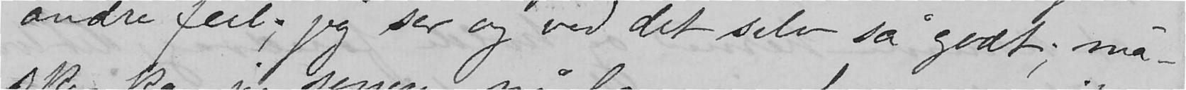andre feil; jeg ser og ved det selv så godt; må-
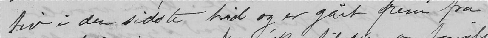fiv i den sidste tid og er gået frem fra
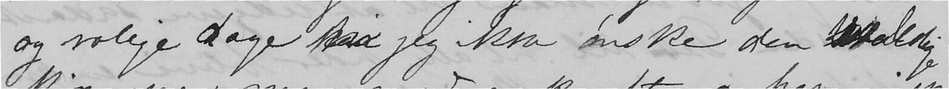og rolige dage kan jeg ikke ønske den statelige
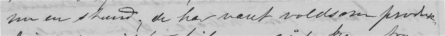nu en stund, de har været voldsom produk-
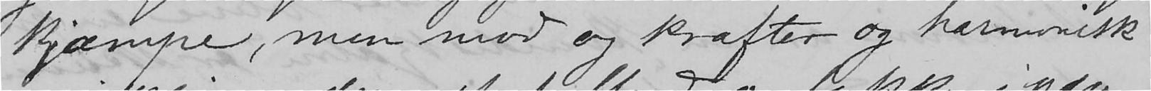kjæmpe, men mod og kræfter og harmonisk
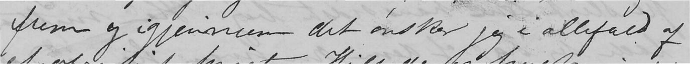frem og igjennem det ønsker jeg i allefald af
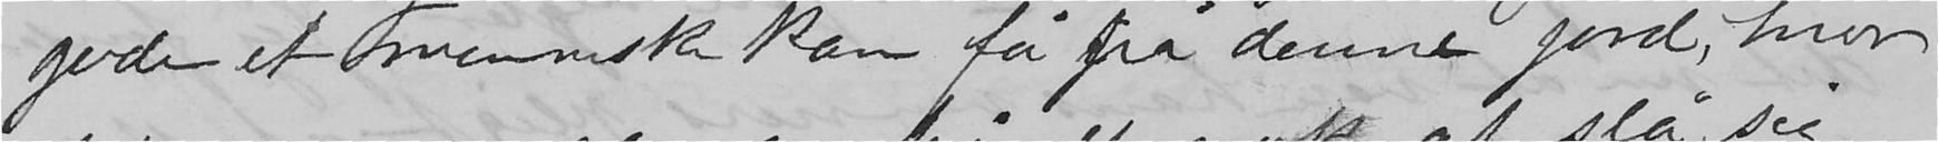gode et menneske kan få fra denne jord, hvor
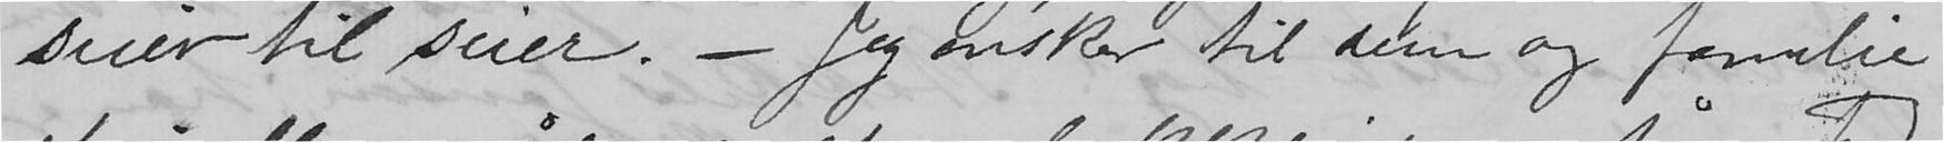seier til seier. - Jeg ønsker til dem og familie
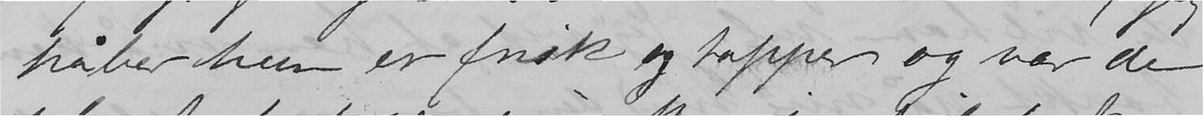håber hun er frisk og tapper, og vær de
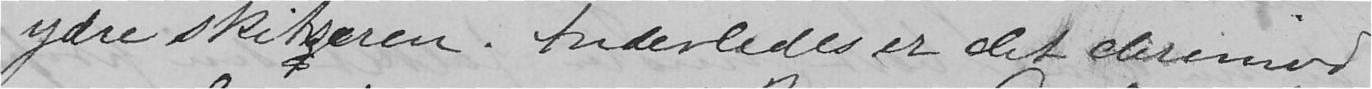ydre skitseren. Anderledes er det derimod
